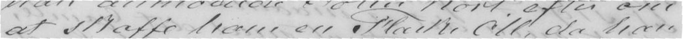at skaffe ham en Flaske Øll, da han
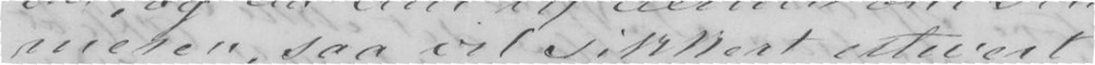meren, saa vil sikkert ethvert
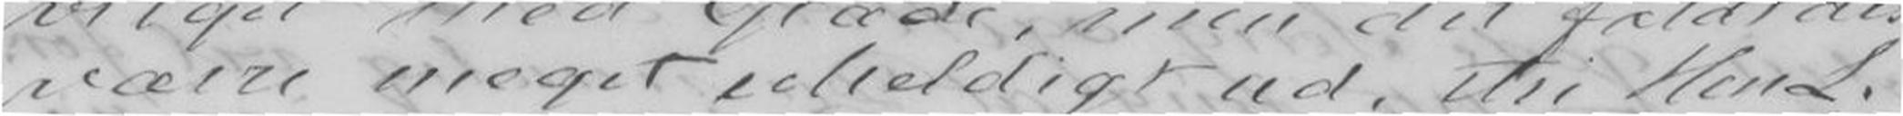værre meget uheldigt ud, thi Herr L.
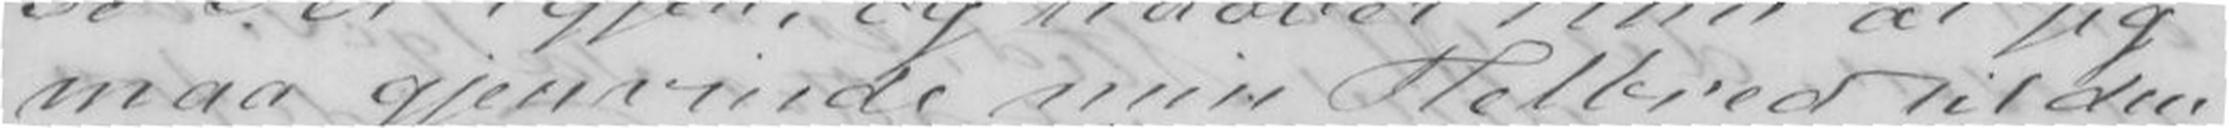maa gjenvinde min Helbred til den
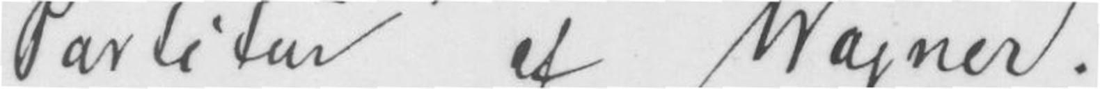Partitur af Wayner.
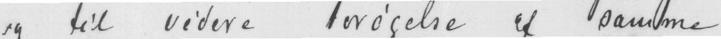og til videre forøgelse af samme
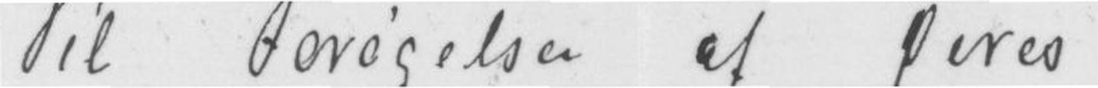Til forøgelsen af Deres
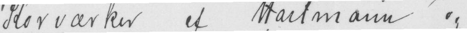Korværker af Hartmann og
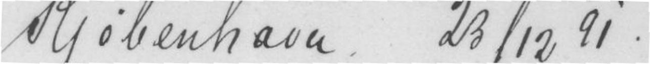Kjøbenhavn 23/12 91
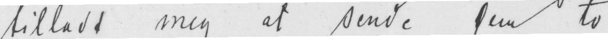tilladt mig at sende dem to
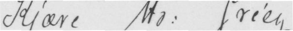Kjære Hr. Grieg
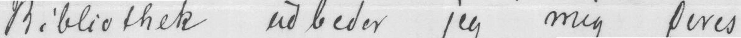Bibliothek udbeder jeg mig Deres
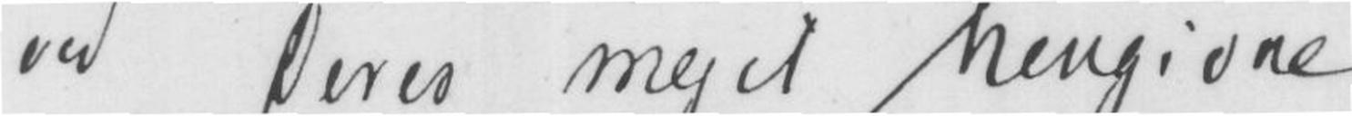ved Deres meget hengivne
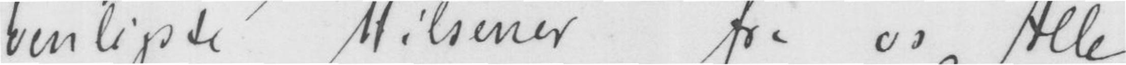venligste Hilsener fra os Alle
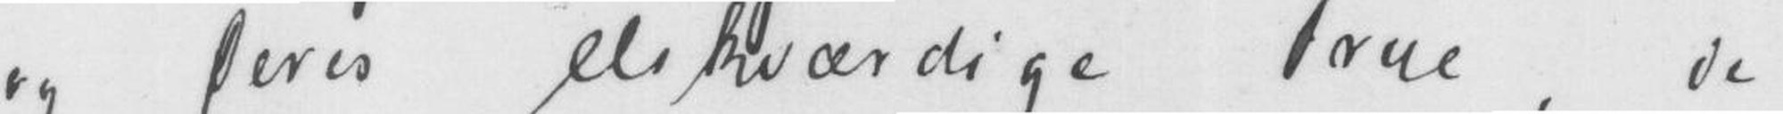og Deres elskværdige frue, De
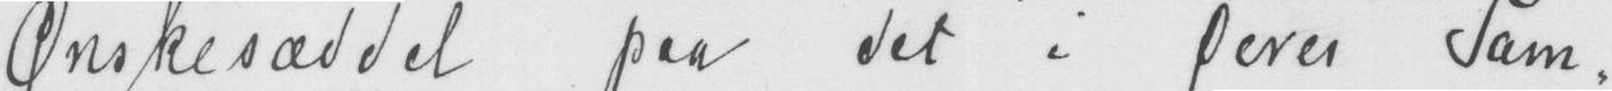Ønskesæddel paa det i Deres Sam-
